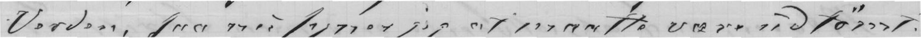Verden saa nu synes jeg at maatte være udtømt.
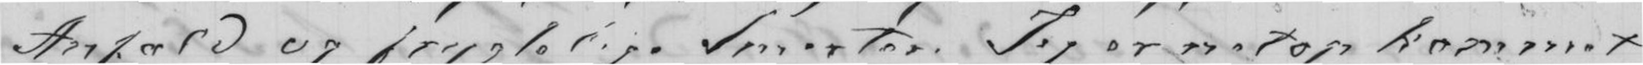Anfald og frygtelig Smerter. Jeg er netop kommet
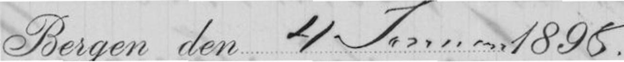Bergen den 4 Januar 1895.
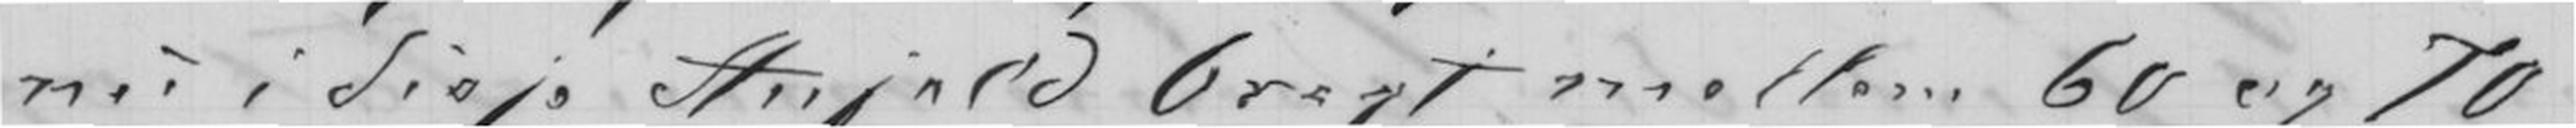nu i disse Anfald bragt mellem 60 og 70
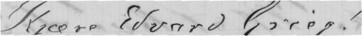Kjære Edvard Grieg!
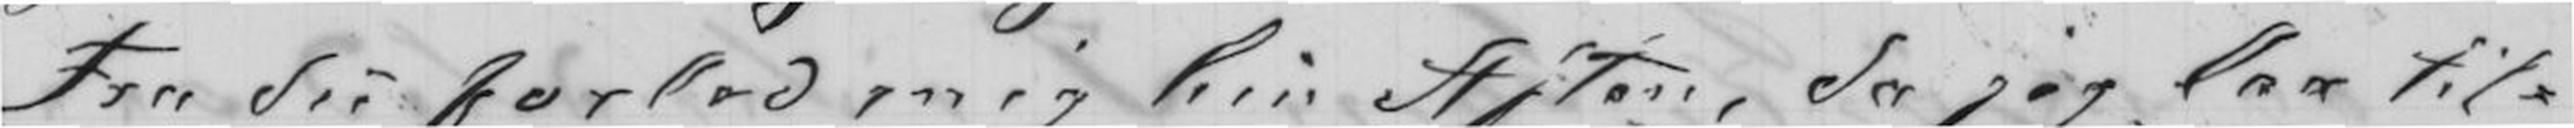Fra Du forlod mig hin Aften, da jeg laa til-
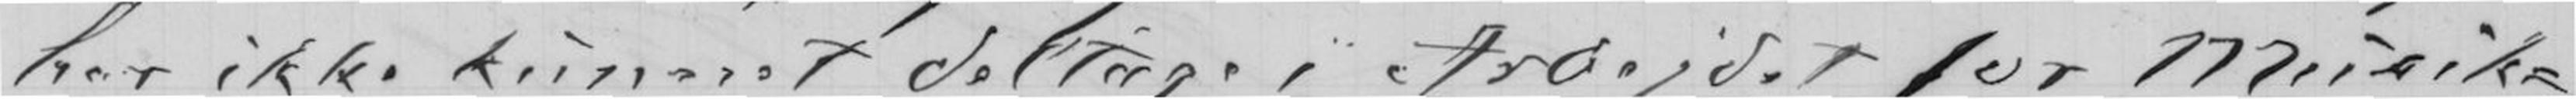har ikke kunnet deltage i Arbejdet for Musik-
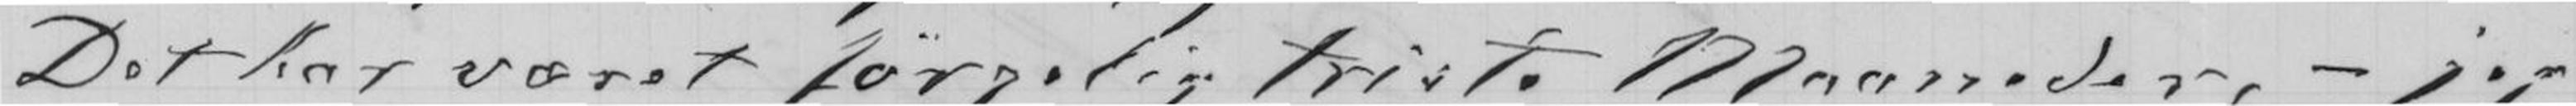Det har været sørgelig triste Maaneder, - jeg
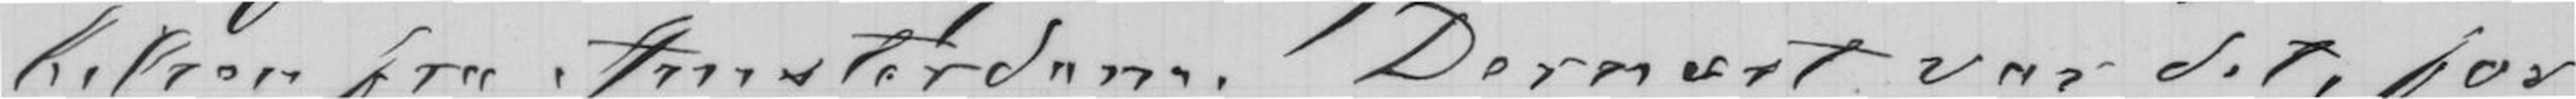hilsen fra Amsterdam. Denæst var det, for
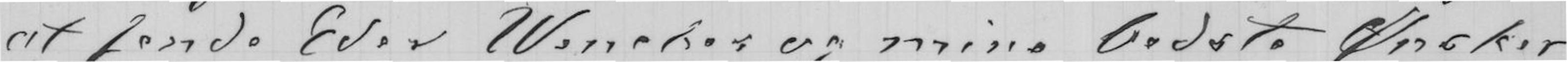at sende Eder Wenches og mine bedste Ønsker
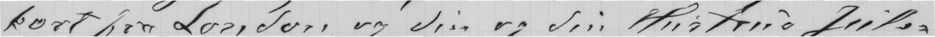kort fra London, og din og din Hustru's Jule-
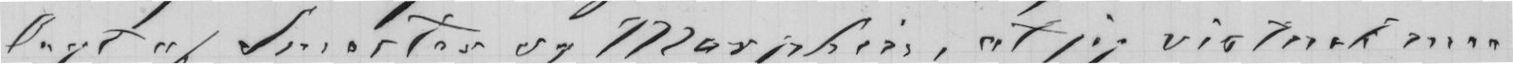lagt af Smerter og Morphin, at jeg vistnok maa
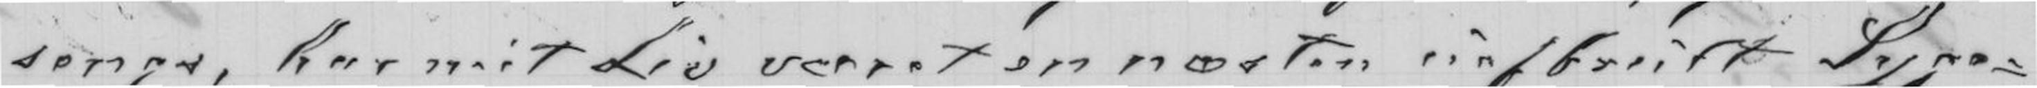sengs, har mit Liv været en næsten uafbrudt Syge-
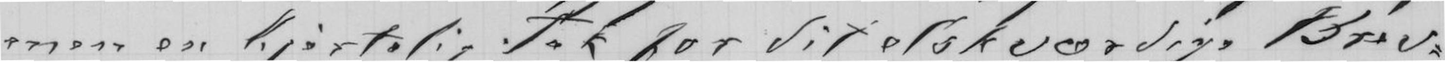men en hjertelig Tak for dit elskværdige Brev-
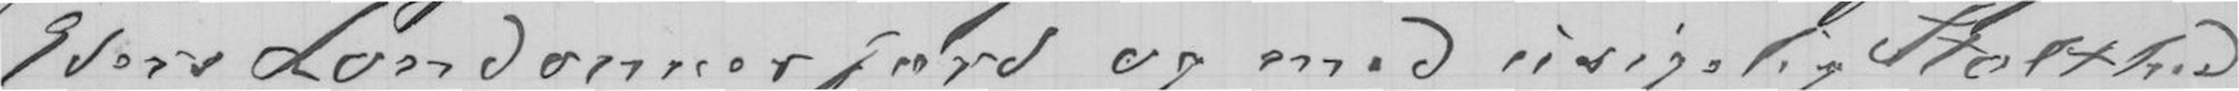Eders Londonnerfærd og med usigelig Stolthed
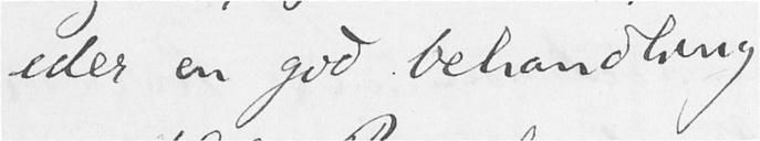eder en god behandling.
